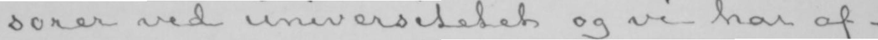sorer ved universitetet og vi har of-
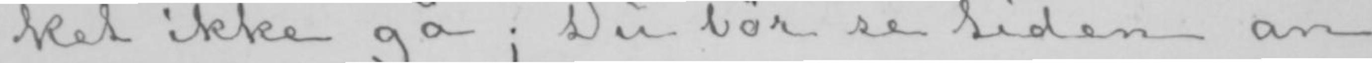ket ikke gå; Du bør se tiden an
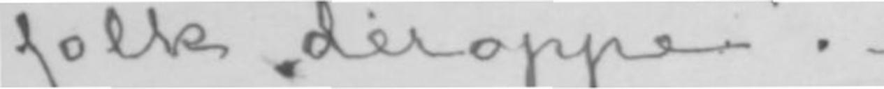folk deroppe.-
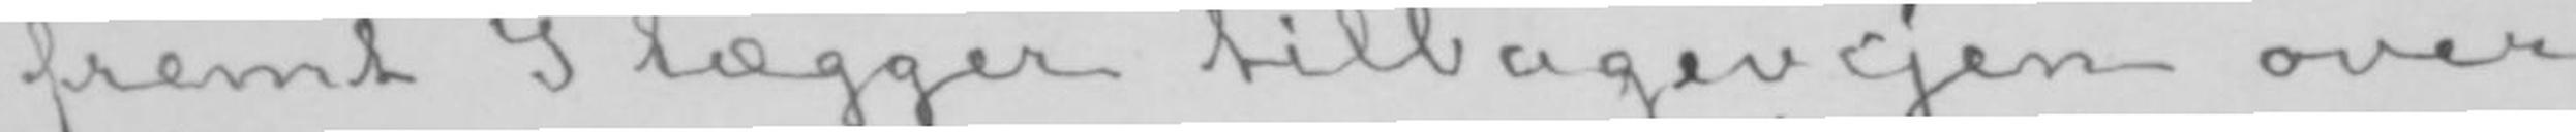fremt I lægger tilbagevejen over
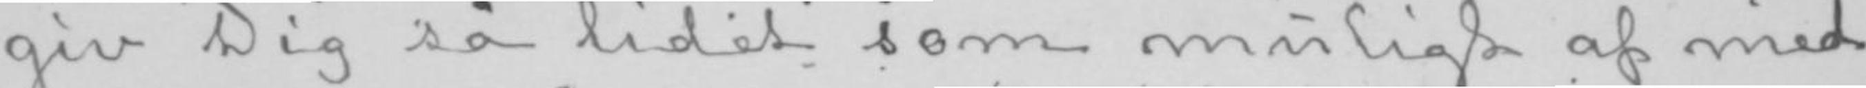giv Dig så lidet som muligt af med
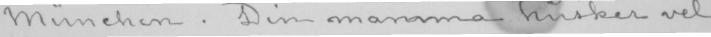München. Din mamma husker vel
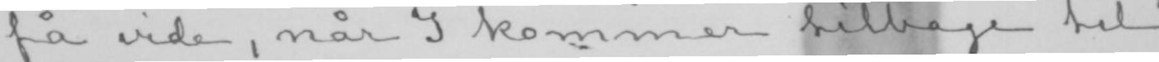få vide, når I kommer tilbage til
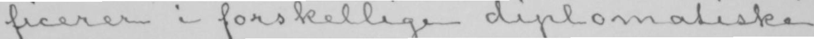ficerer i forskellige diplomatiske
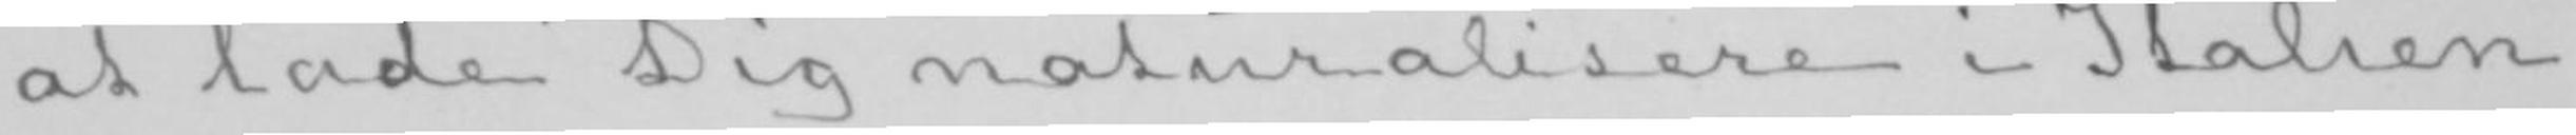at lade Dig naturalisere i Italien
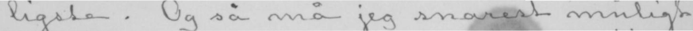ligste. Og så må jeg snarest muligt
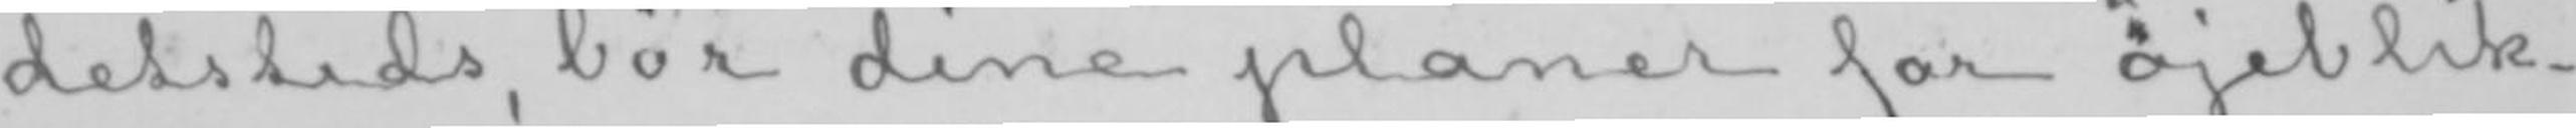detsteds, bør dine planer for øjeblik-
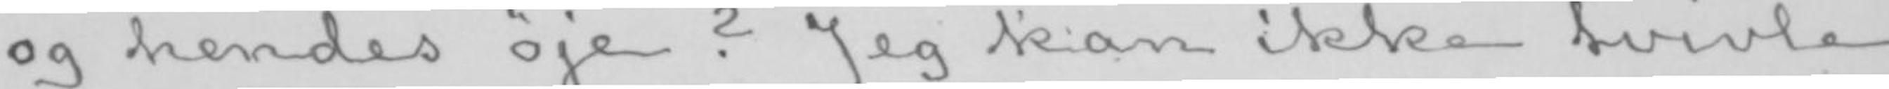og hendes øje? Jeg kan ikke tvivle
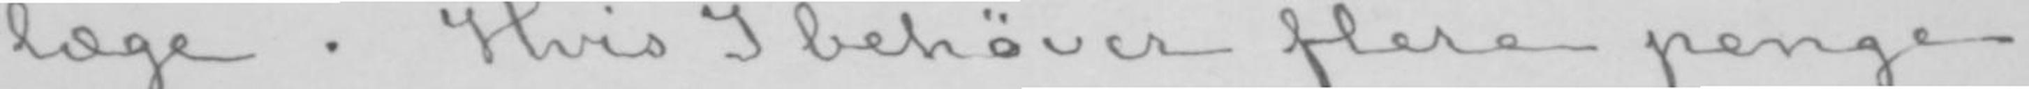læge. Hvis I behøver flere penge,
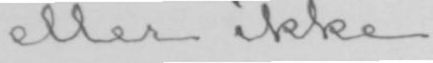eller ikke.
